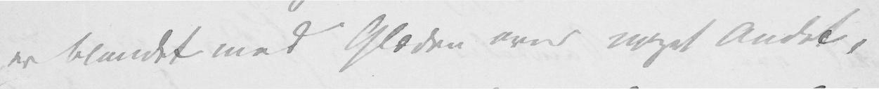er blandet med Glæden over meget Andet,
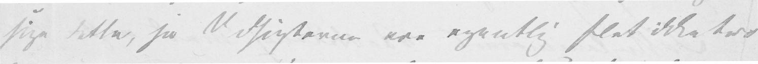sige dette, ja Udsigterne ere egentlig slet ikke trø-
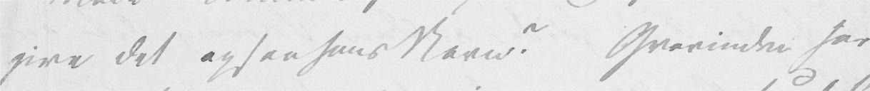give det ogsaa hans Navn? Grevinden har
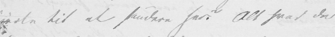Hjerte til at sondere her? Alt hvad der
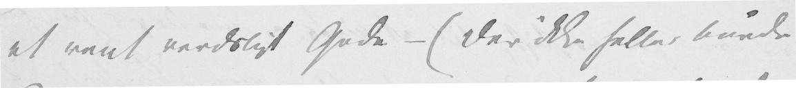et rent verdsligt Gode – (der ikke heller burde
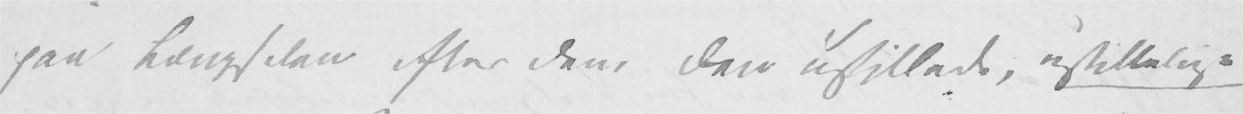paa Længselen efter den, den ustillede, ustillelige
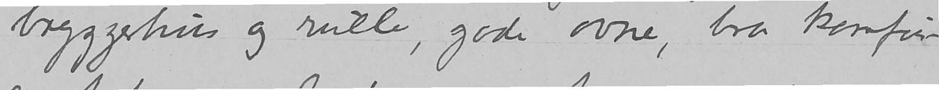bryggerhus og rulle, gode ovne, bra komfyr
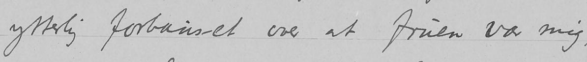ytterlig forbauset over at fruen var mig,
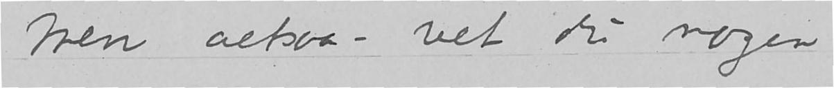Men altsaa – vet du nogen
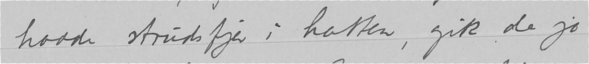hadde strudsfjer i hatten, gik de jo
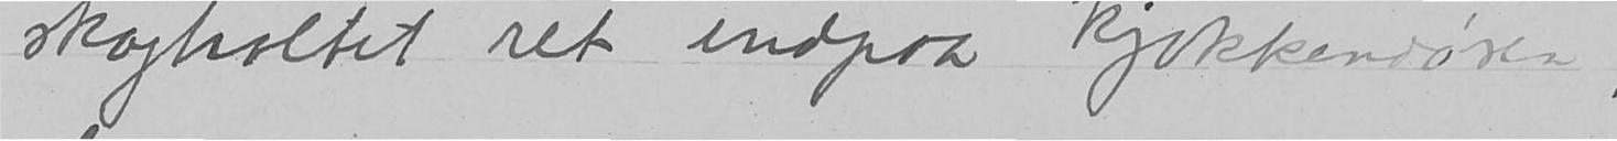skogholtet ret indpaa kjøkkendøren,
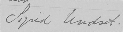Sigrid Undset.
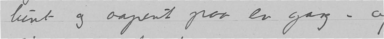lunt og aapent paa en gang – og
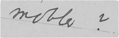møbler?
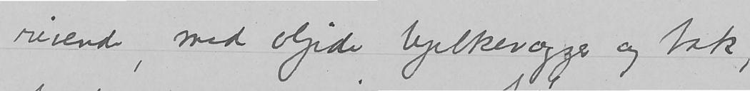rivende med oljede bjelkevægger og tak,
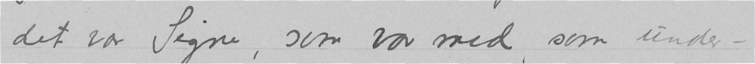det var Signe, som var med, som under –
